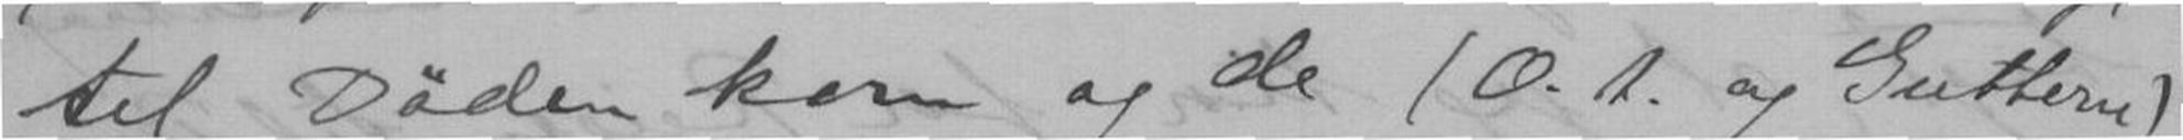til Døden kom og de (O. A. og Guttorne)
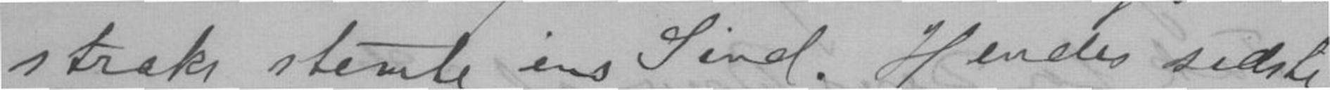straks stemte ens Sind. Hendes sidste
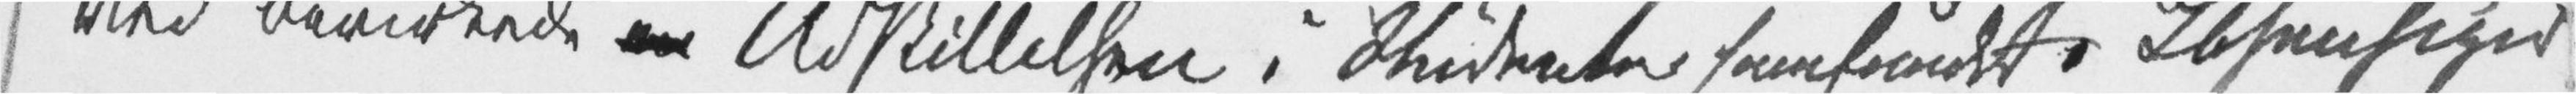ved bevirkede en Adskillelsen i Studentersamfundet. Ibsen siger
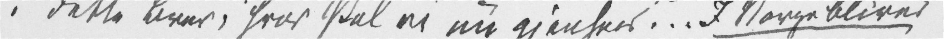i dette Brev, «hvor skal vi nu gjensees?.. I Norge bliver
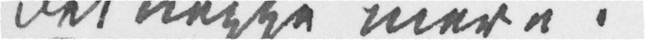det neppe mere».
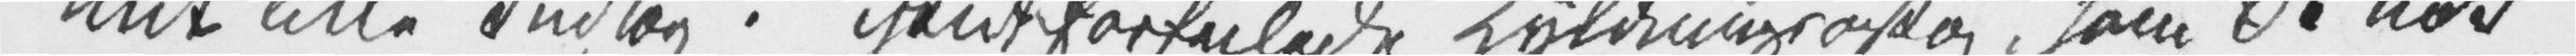mit lille Indlæg i hint forfeilede Hyldningsoptog, som De nok
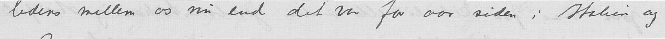ledes mellem os nu end det var for aar siden i Italien og
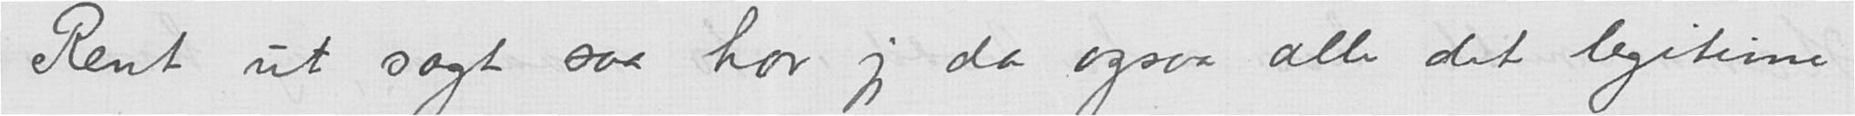Rent ut sagt saa har jeg da ogsaa alle det legitime
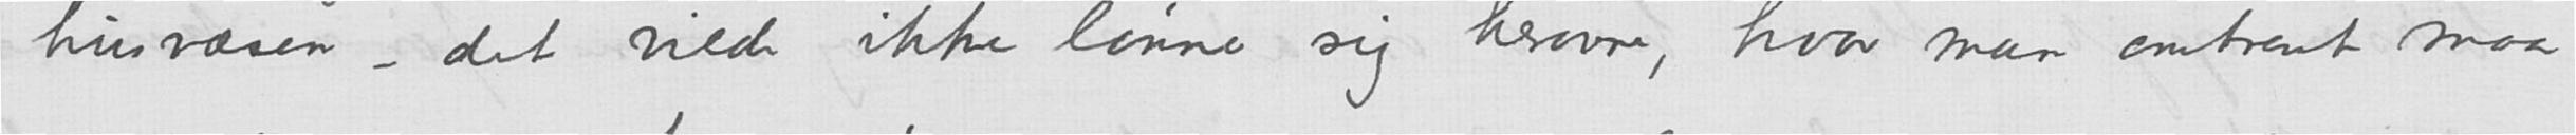husvæsen - det vilde ikke lønne sig herovre, hvor man omtrent maa
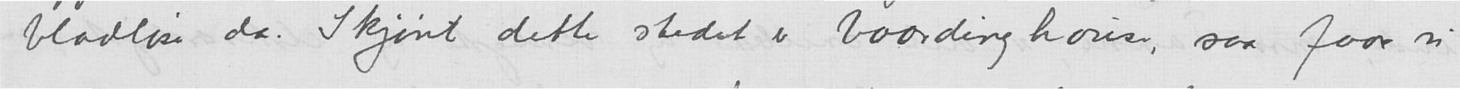bladløse da. Skjønt dette stedet er boardinghouse, saa faar vi
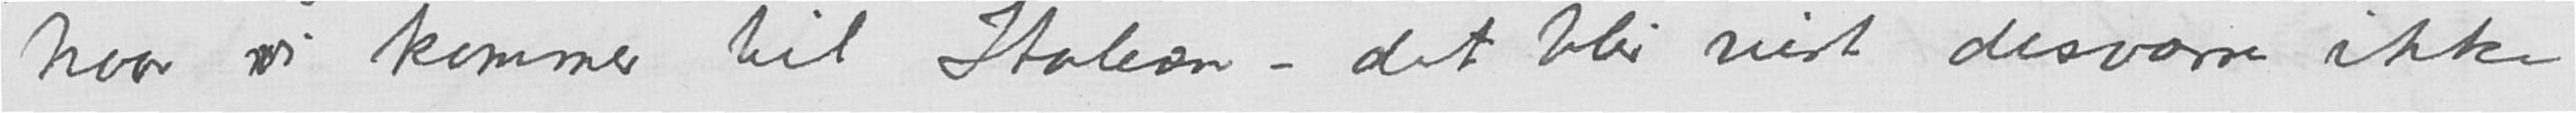Naar vi kommer til Italien - det blir vist desværre ikke
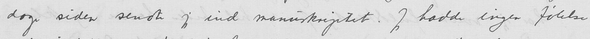dager siden sendte jeg ind manuskriptet. Jeg hadde ingen følelse
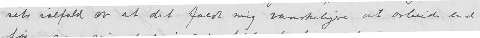selv ialfald av at det faldt mig vanskeligere at arbeide end
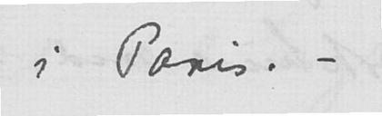i Paris. -
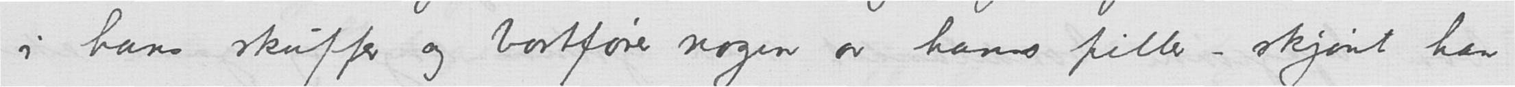i hans skuffer og bortfører nogen av hans filler - skjønt han
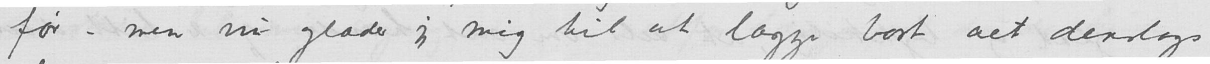før - men nu glæder jeg mig til at lægge bort alt denslags
